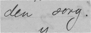den sorg.
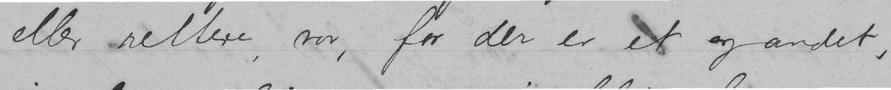eller rettere, var, for der er et og andet,
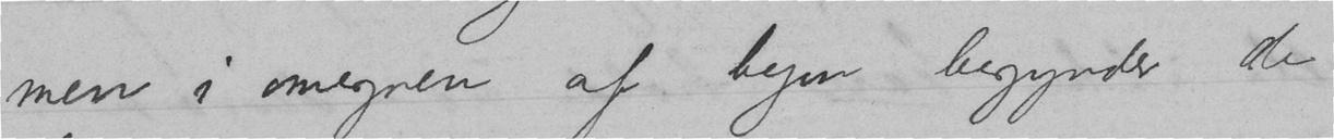men i omegnen af byen begynder de
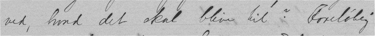ved, hvad det skal blive til? Foreløbig
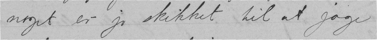noget er jo skikket til at jage
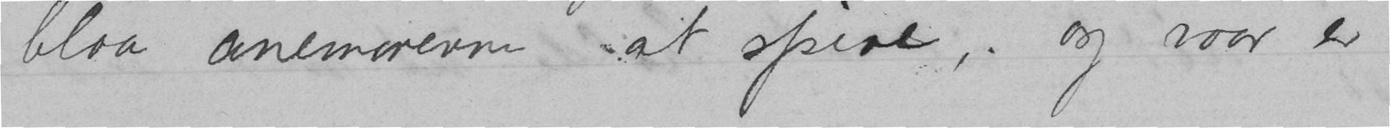blaa anemonerne at spire, og vaar er
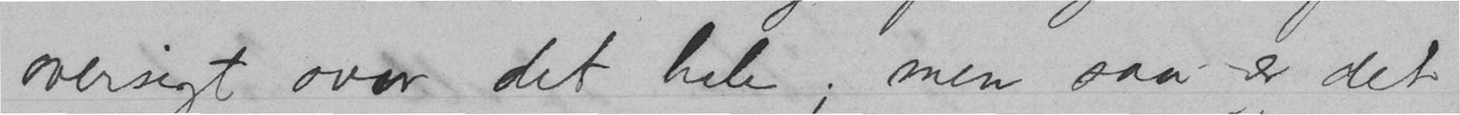oversigt over det hele; men saa er det
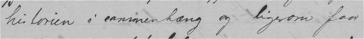historien i sammenhæng og ligesom faar
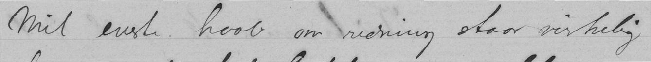Mit eneste haab om redning staar virkelig
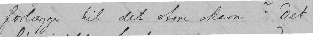forlægger til det store skam? Det
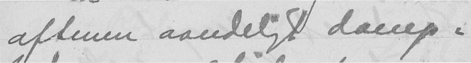aftenen aandeligt damp-
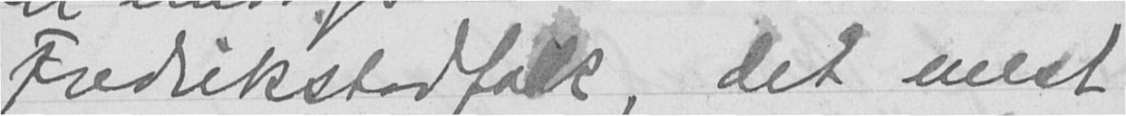Fredrikstadfolk, det mest
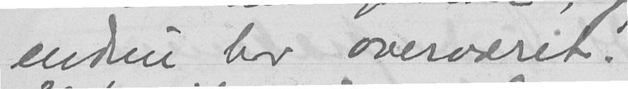endnu har overværet.
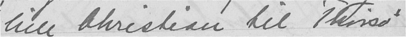lille Christian til Thorsø
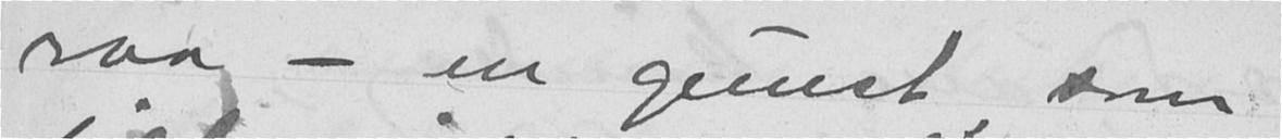raa - en gevinst som
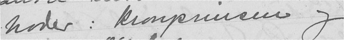hoder: kronprinsen og
